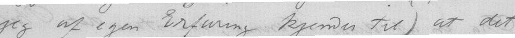jeg af egen Erfaring kjender til) at det
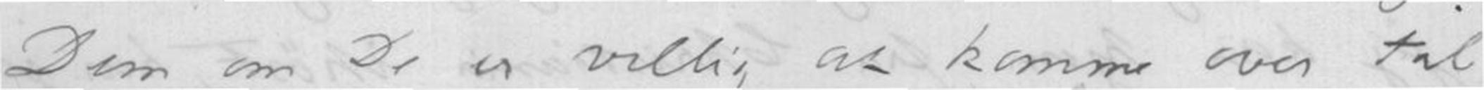Dem, om De er villig at komme over til
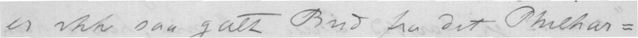er ikke saa galt Bud fra det Philhar-
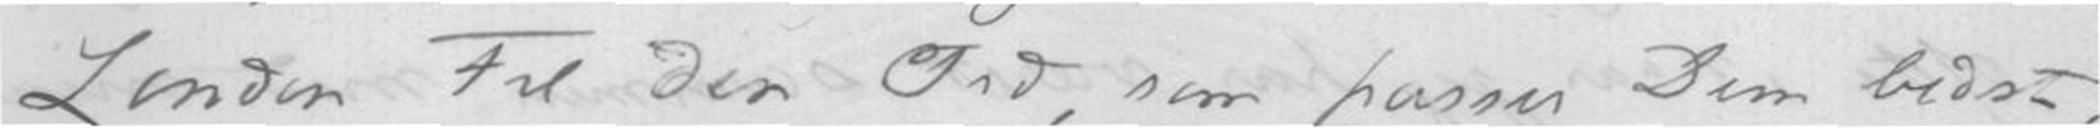London til den Tid, som passer Dem bedst,
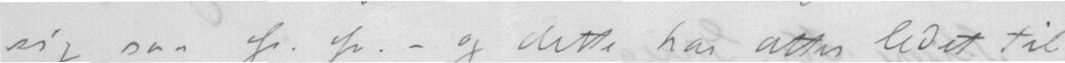sig saa osv. osv. - og dette har atter lidet til
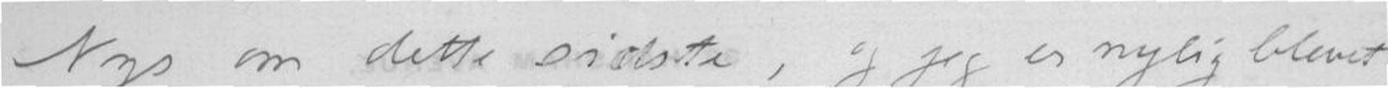Nys om dette sidste, og jeg er nylig blevet
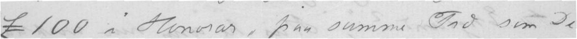£100 i Honorar, paa samme Tid som De
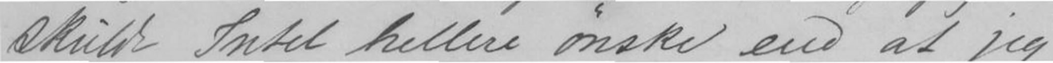skulde Intet hellere ønske end at jeg
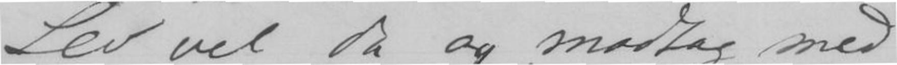Lev vel da og modtag med
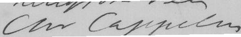Chr. Cappelen
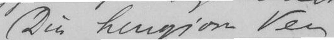Din hengivne Ven
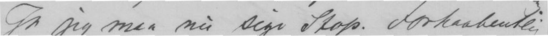Gi jeg maa nu sige Stop. Forhaabentlig
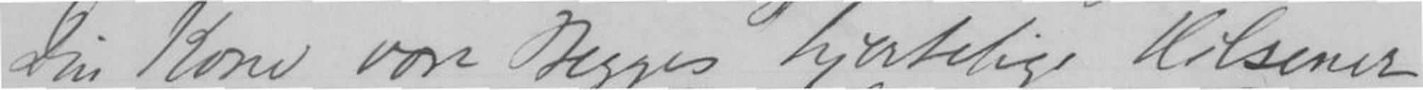Din Kone vore Begges hjertelige Hilsener
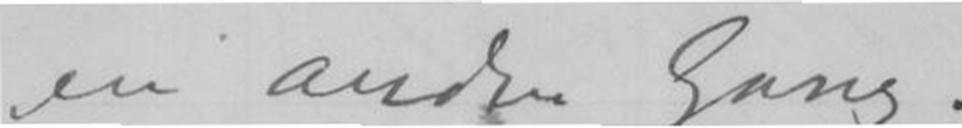en anden Gang.
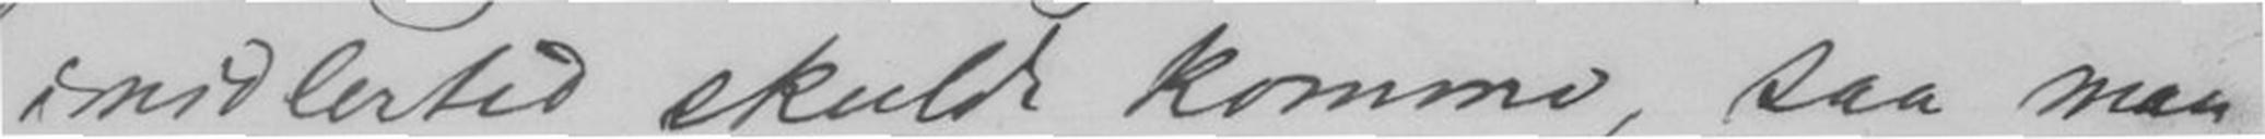imidlertid skulde komme, saa maa
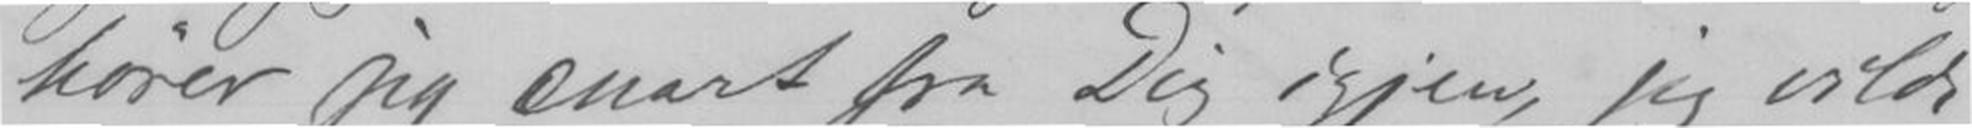hører jeg snart fra Dig igjen, jeg vilde
